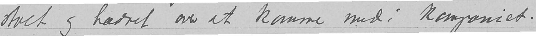stolt og hædret over at komme med i kompaniet.
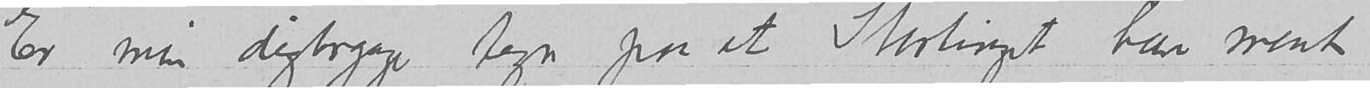Er min digtergage tegn paa at Stortinget har ment
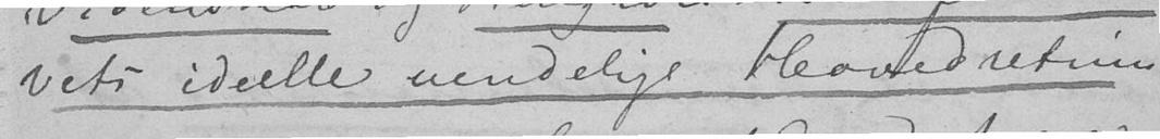vets ideelle uendelige Hovedretnin-
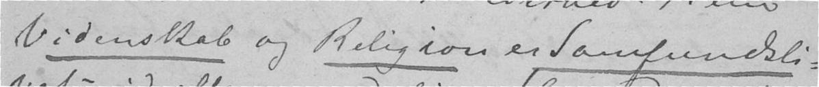Videnskab og Religion er Samfundsli-
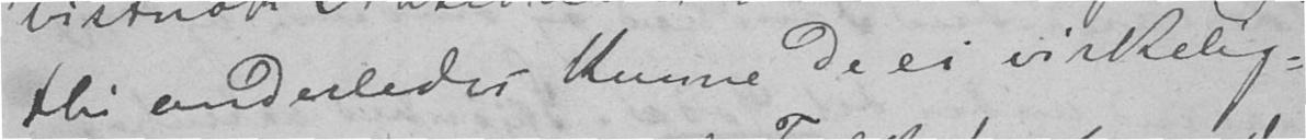thi anderledes kunne de ei virkelig-
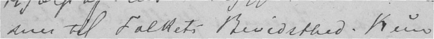mer til Folkets Bevidsthed. Kun
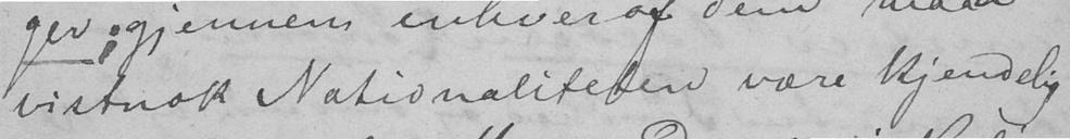vistnok Nationaliteten være kjendelig;
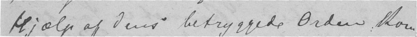Hjælp af dens betryggede Orden kom-
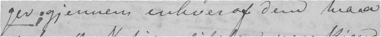ger; gjennem enhver af dem maa
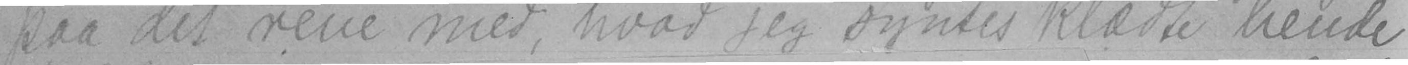paa det rene med, hvad seg syntes klædte hende
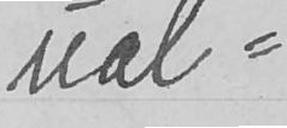ual-
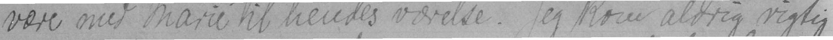være med Marie til hendes værelse. Jeg kom aldrig rigtig
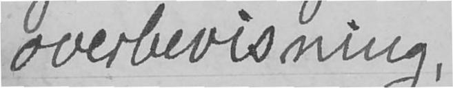overbevisning,
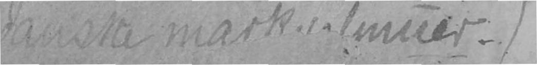danske marksvalmuer.)
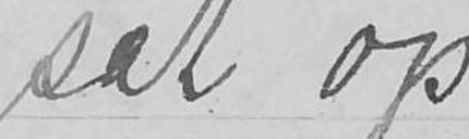sat op
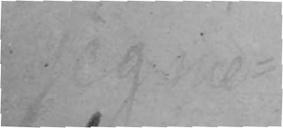jeg me-
